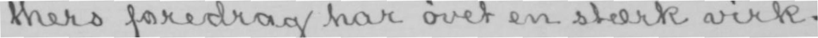thers foredrag har øvet en stærk virk-
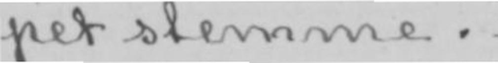pet stemme.-
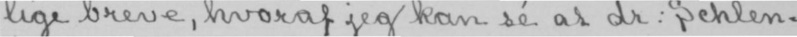lige breve, hvoraf jeg kan sé at dr: Schlen-
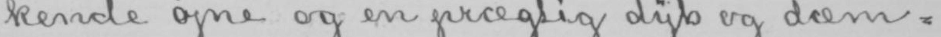kende øjne og en prægtig dyb og dæm-
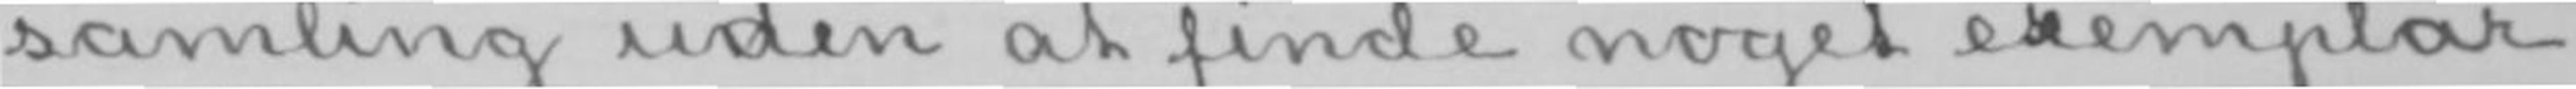samling uden at finde noget exemplar
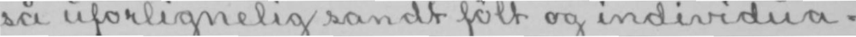så uforlignelig sandt følt og individua-
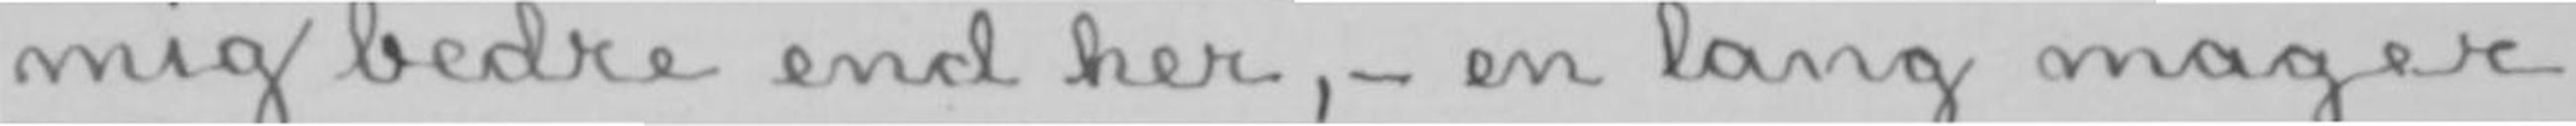mig bedre end her,- en lang mager
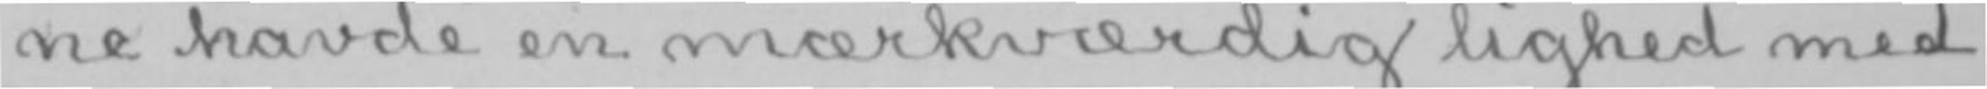ne havde en mærkværdig lighed med
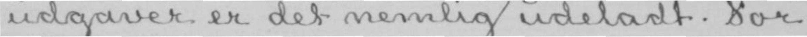udgaver er det nemlig udeladt. For
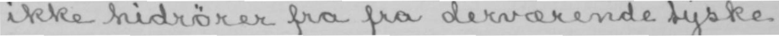ikke hidrører fra fra derværende tyske
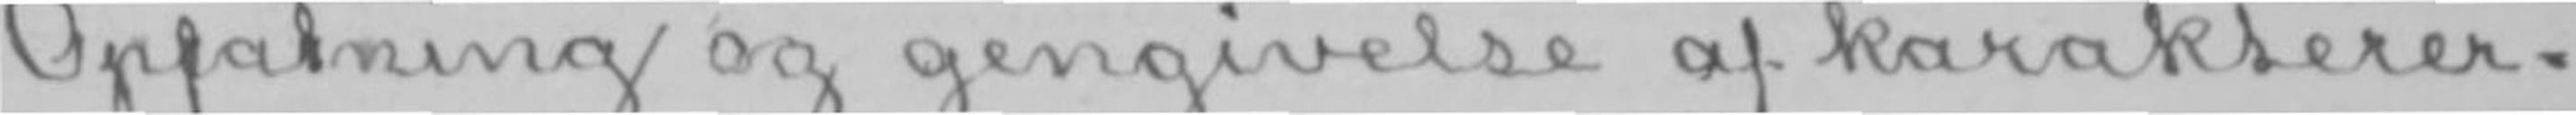Opfatning og gengivelse af karakterer-
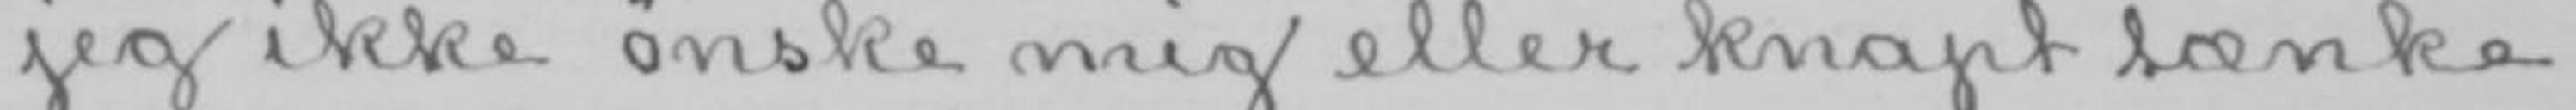jeg ikke ønske mig eller knapt tænke
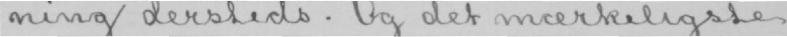ning dersteds. Og det mærkeligste
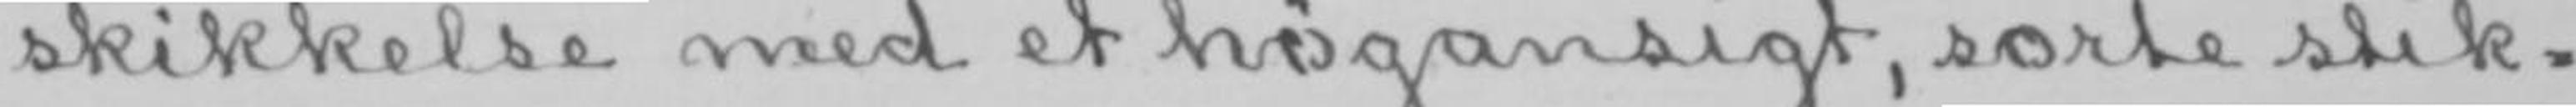skikkelse med et høgansigt, sorte stik-
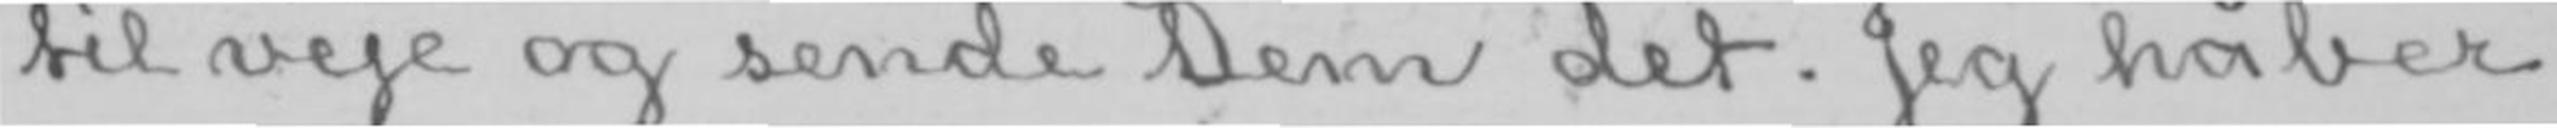til veje og sende Dem det. Jeg håber
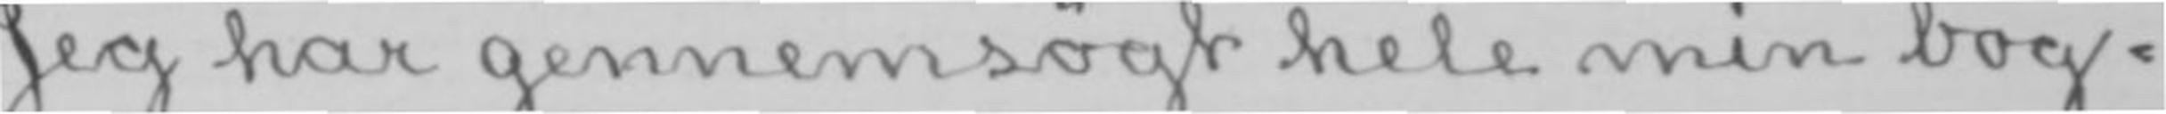Jeg har gennemsøgt hele min bog-
